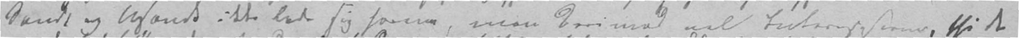Sandt og Usandt ikke lade sig forene, men derimod vel Interesserne, thi de
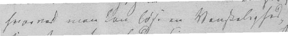hvorved man kan løse en Vanskelighed,
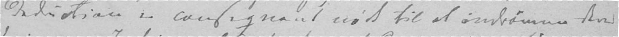Deduction er conseqvent nødt til at indrømme dem
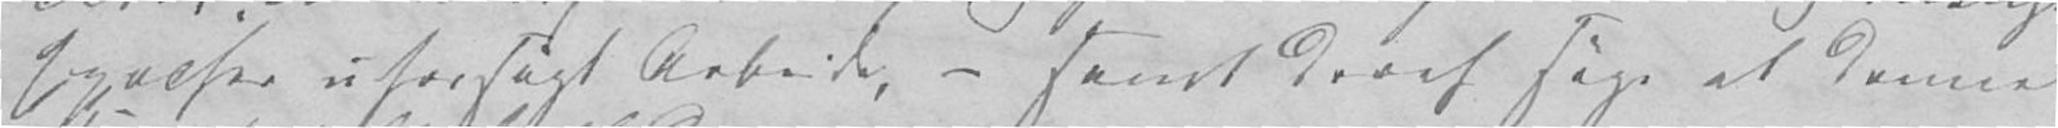Epocher uforsøgt Arbeide, – samt deraf søge at danne
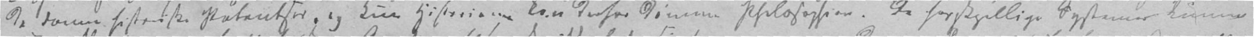de danne historiske Potentser, og kun Historien kan derfor dømme Philosophien. De forskjellige Systemer kunne
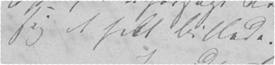sig et helt Billede.
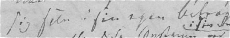sig selv i sin egen Betragtning, og at al Sikkerhed i Forskningens Resultat paa Forhaand maatte opgives
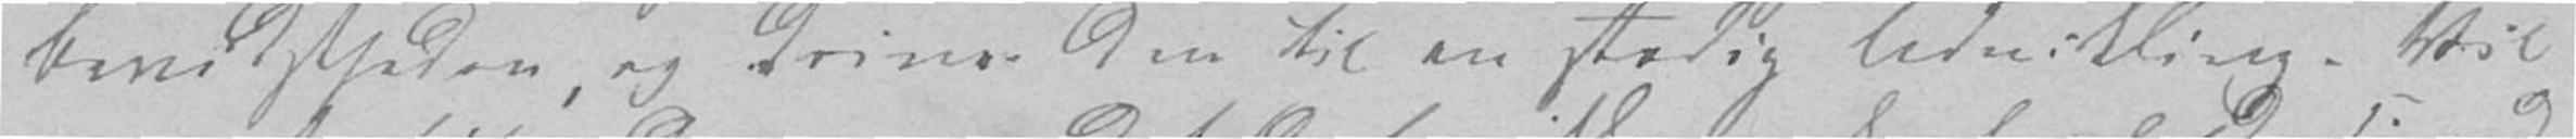bevidstheden, og driver den til en stadig Udvikling. Vil
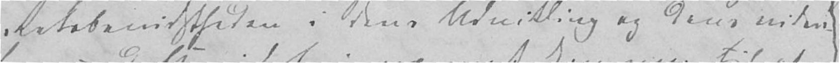Retsbevidstheden i dens Udvikling og dens viden-
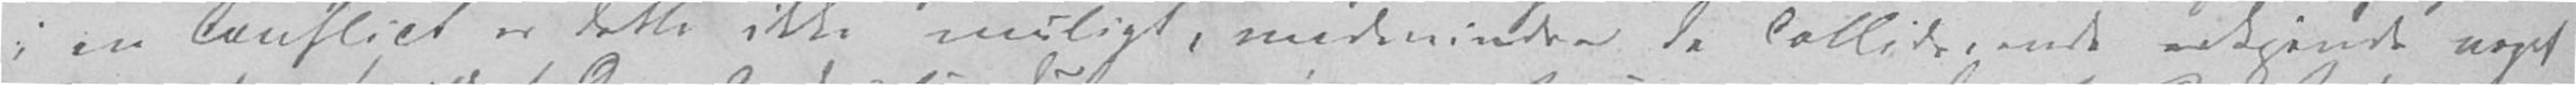i en Conflict er dette ikke muligt, medmindre de colliderende erkjende noget
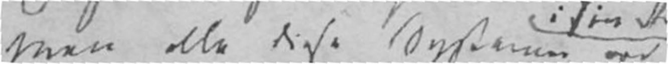Men alle disse Systemer ere
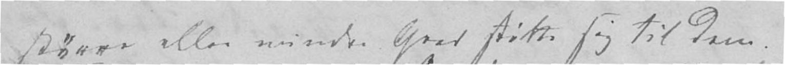større eller mindre Grad støtte sig til dem.
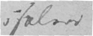isolere
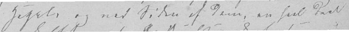Hegels og ved Siden af dem, en heel Deel
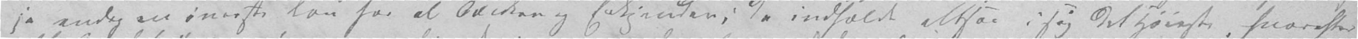ja endog en øverste Lov for al Tænken og Erkjenden, de indholde altsaa i sig det Høieste, hvorefter
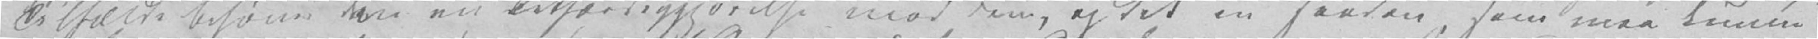Tilfælde behøver den en Retfærdiggjørelse mod dem, og det en saadan, som maa kunne
